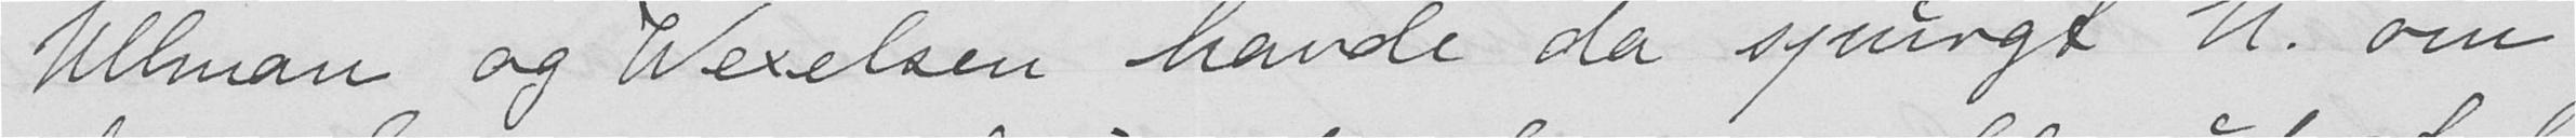Ulman og Wexelsen havde da spurgt N. om
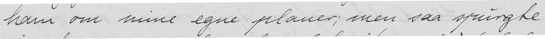ham om mine egne planer; men saa spurgte
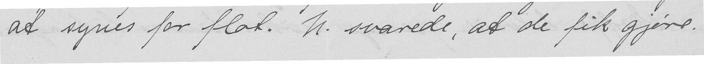at synes for flot. N. svarede, at de fik gjøre.
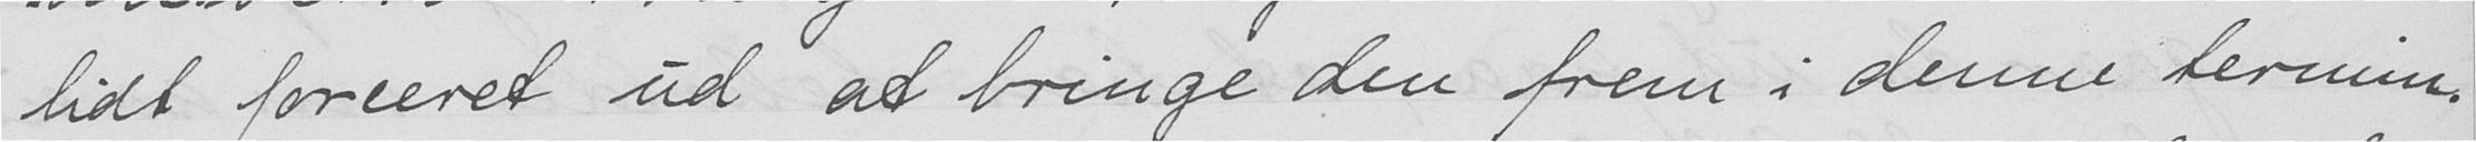lidt forceret ud at bringe den frem i denne termin.
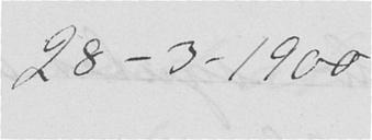28-3-1900
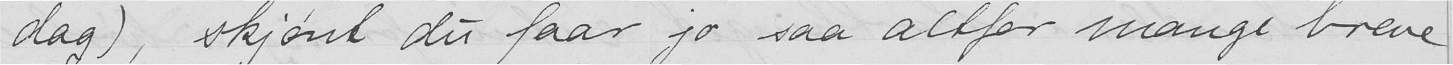dag), skjønt du faar jo saa altfor mange breve
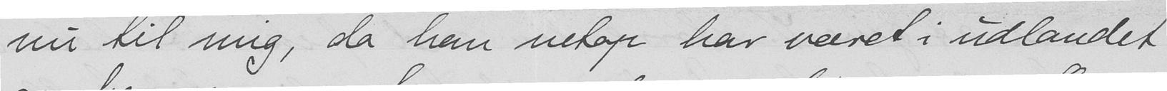nu til mig, da han netop har været i udlandet
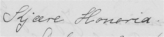Kjære Honoria!
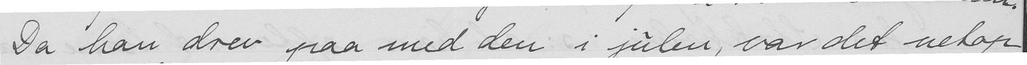Da han drev paa med den i julen, var det netop
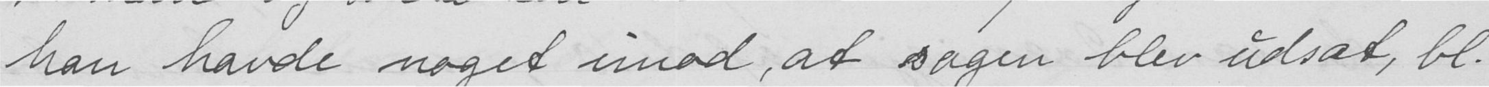han havde noget imod, at sagen blev udsat, bl.
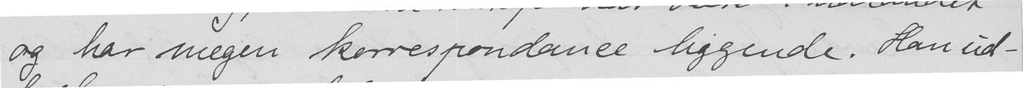og har megen korrespondance liggende. Han ud-
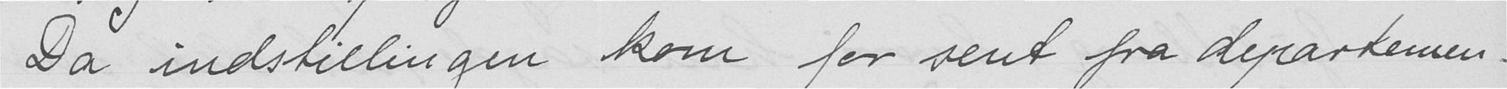Da indstillingen kom for sent fra departemen-
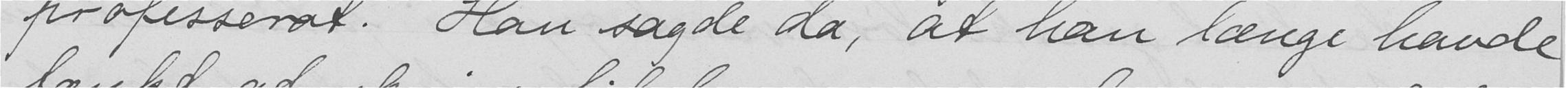professorat. Han sagde da, at han længe havde
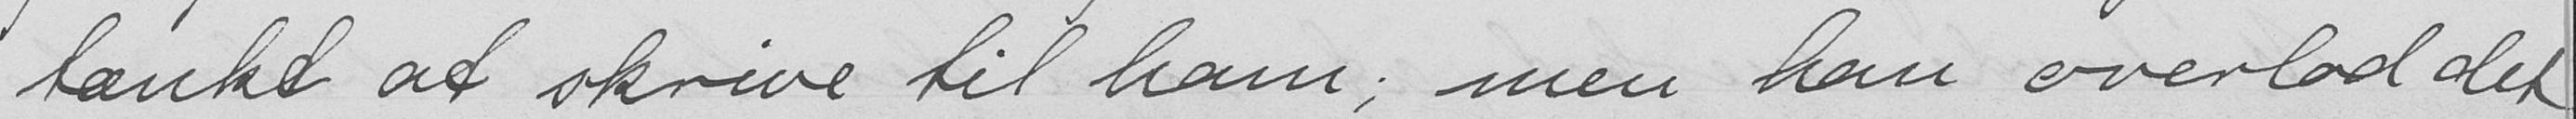tænkt at skrive til ham; men han overlod det
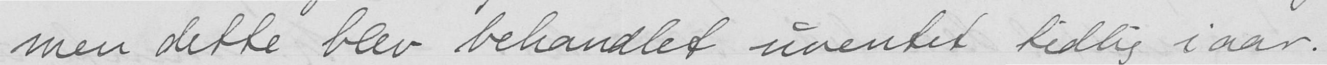men dette blev behandlet uventet tidlig iaar.
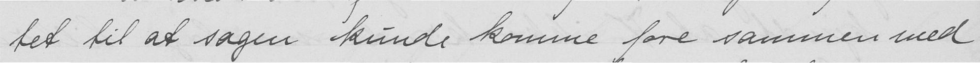tet til at sagen kunde komme fore sammen med
